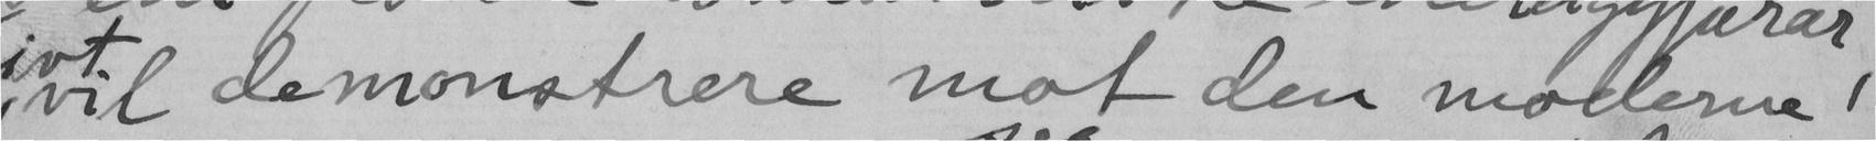vil demonstrere mot den moderne
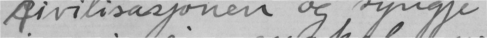sivilisasjonen og syngje
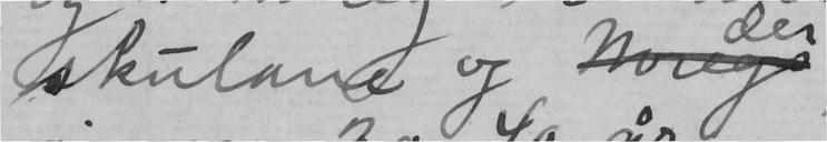skulane og Noregs
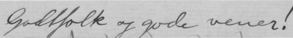Godtfolk og gode vener!
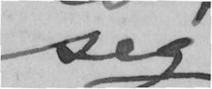seg
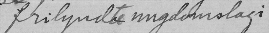frilyndte ungdomslagi
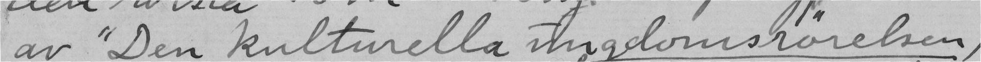av "Den kulturella ungdomsrørelsen,
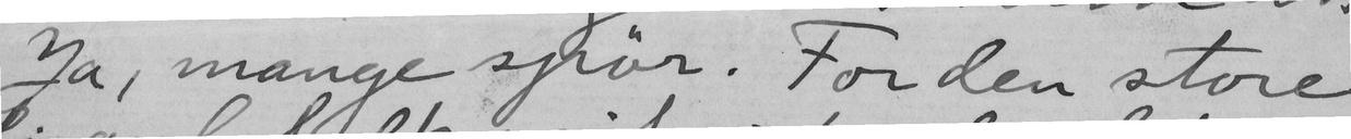Ja, mange spør. For den store
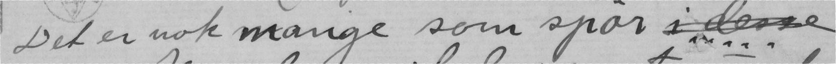Det er nok mange som spør i desse
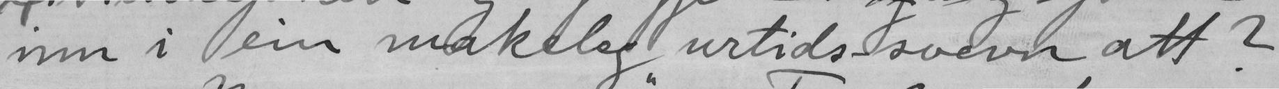inn i ein makeleg urtids-svevn att?
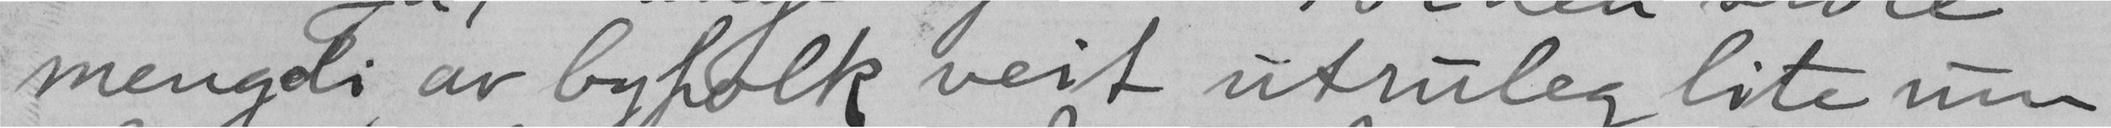mengdi av byfolk veit utruleg lite um
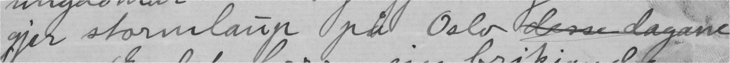gjer stormlaup på Oslo desse dagane?
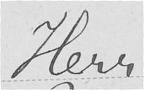Herr
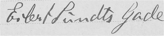Eilert Sundts Gade.
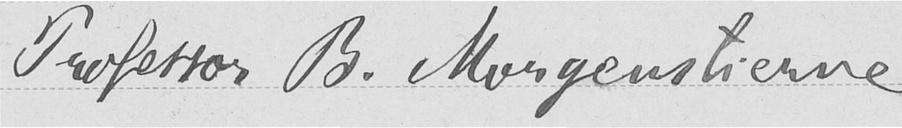Professor B. Morgenstierne
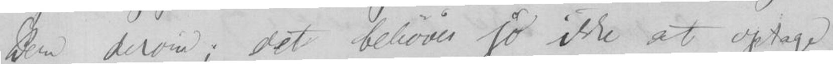Dem derom; det behøver jo ikke at optage
